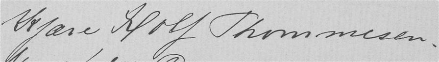Kjære Rolf Thommesen.
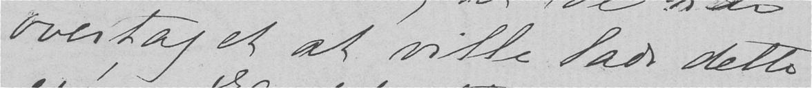overtaget at ville lade dette
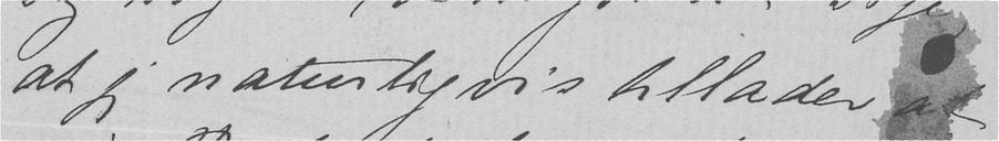at jeg naturligvis tillader at
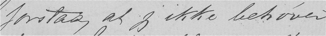forstaa, at jeg ikke behøver
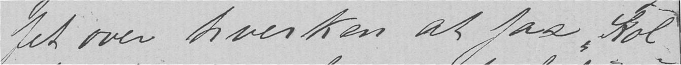fet over hverken at faa "Kol-
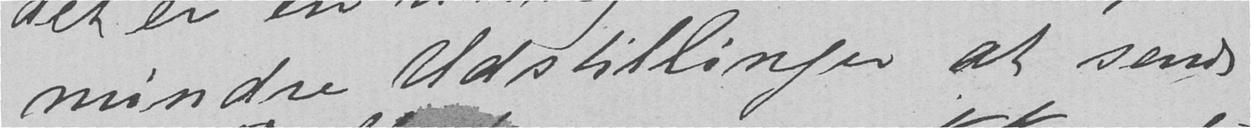mindre Udstillinger at sende
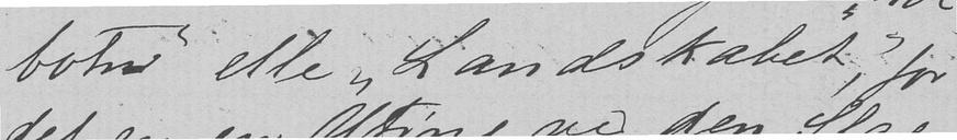botn" eller "Landskabet", for
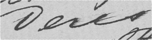Deres
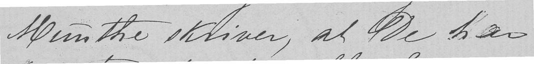Munthe skriver, at De har
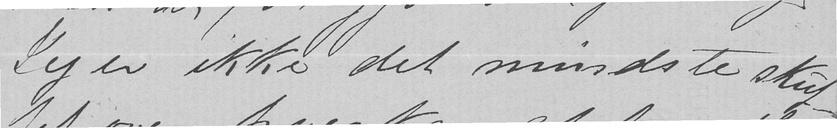Jeg er ikke det mindste skuf-
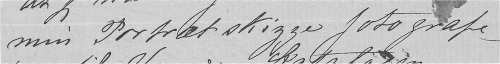min Portrætskizze fotografe-
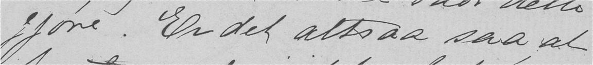gjøre. Er det altsaa saa at
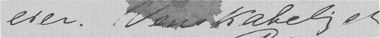eier. Venskabeligst
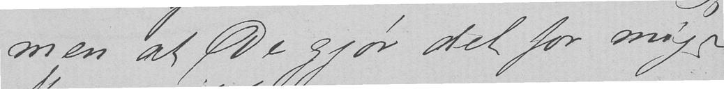men at De gjør det for mig?
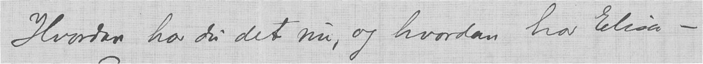Hvordan har du det nu, og hvordan har Elisa-
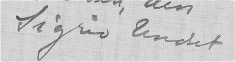Sigrid Undset
